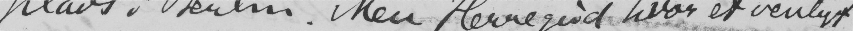plads i Berlin. Men Herregud, hvor et venligt
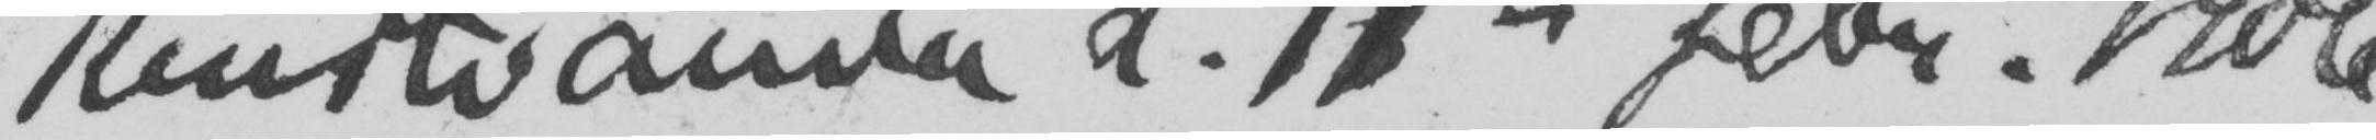Kristiania d. 17de febr. 1906.
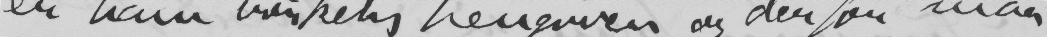er ham virkelig hengiven, og derfor maa
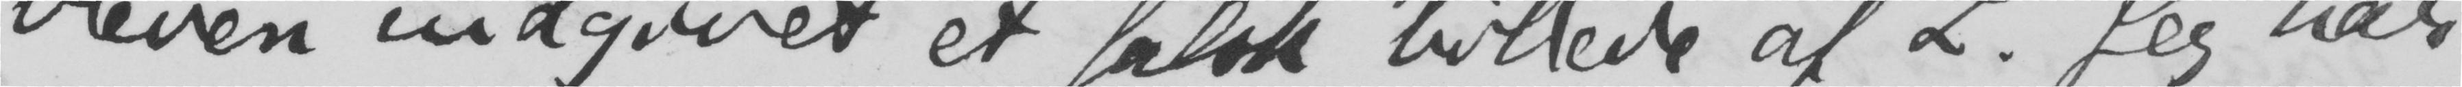bleven indgivet et falsk billede af L. Jeg har
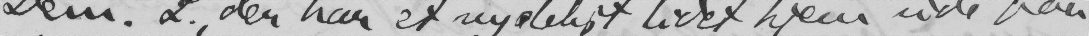Dem. L., der har et nydeligt lidet hjem ude paa
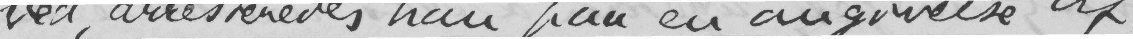ved, arresteredes han paa en angivelse af
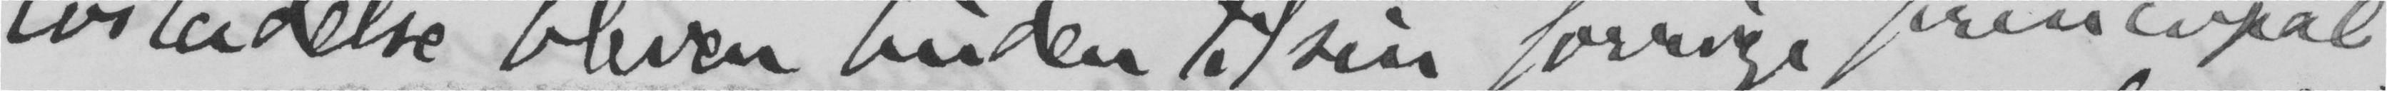løsladelse bleven buden til sin forrige principal
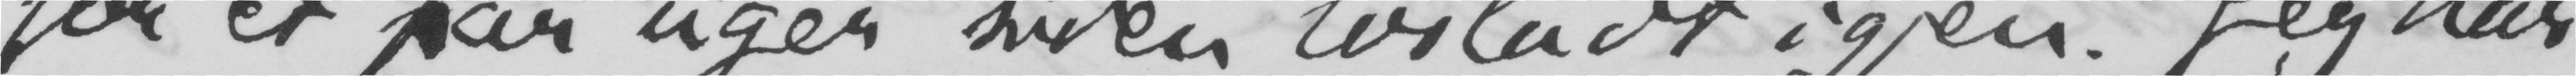for et par uger siden løsladt igjen. Jeg har
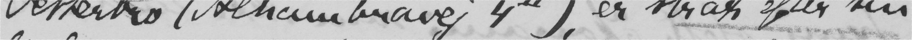Vesterbro (Alhambravej 4II), er strax efter sin
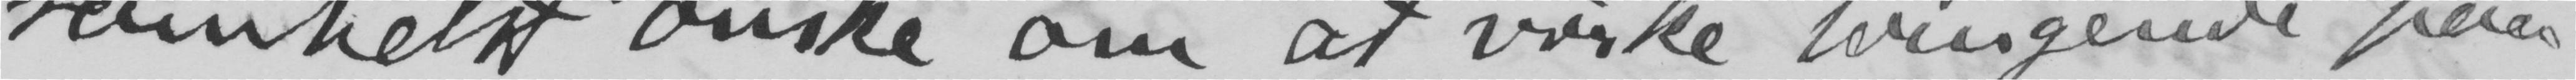somhelst ønske om at virke tvingende paa
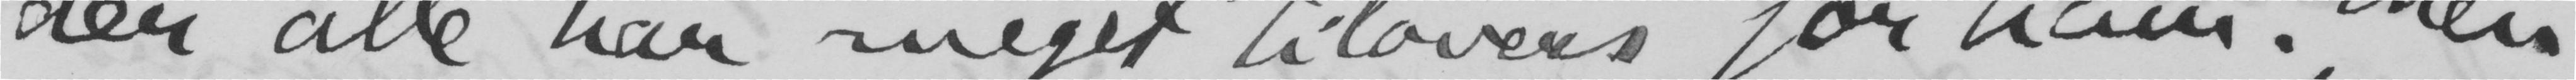der alle har meget tilovers for ham. Men
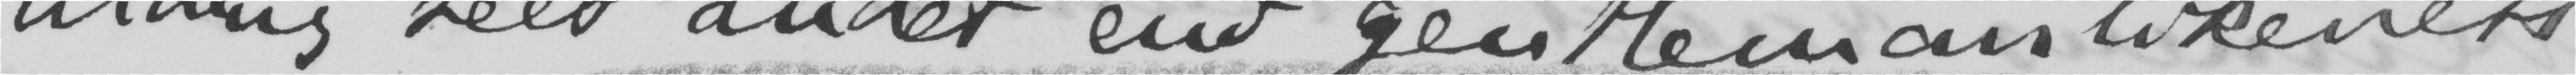aldrig seet andet end gentlemanlikeness
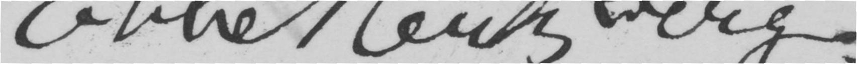Ebbe Hertzberg
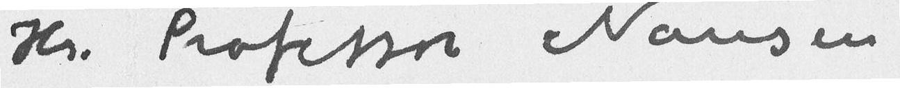Hr. Professor Nansen
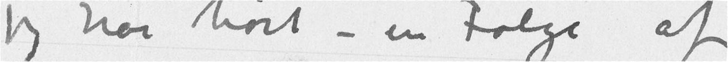jeg har hørt – en Følge af
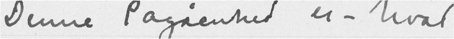Denne Pågåenhed er – hvad
